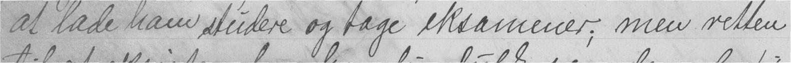at lade ham studere og tage eksamener; men retten
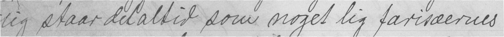lig staar det altid som noget lig farisæernes
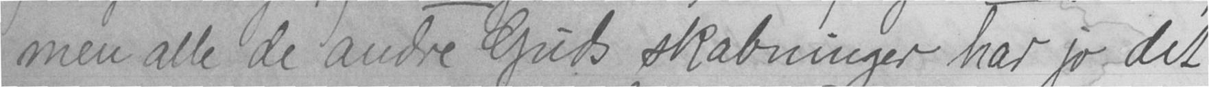men alle de andre Guds skabninger har jo det
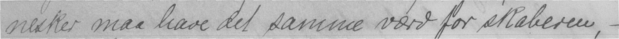nesker maa have det samme værd for skaberen, -
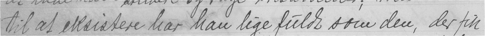til at eksistere har han lige fuldt som den, der fik
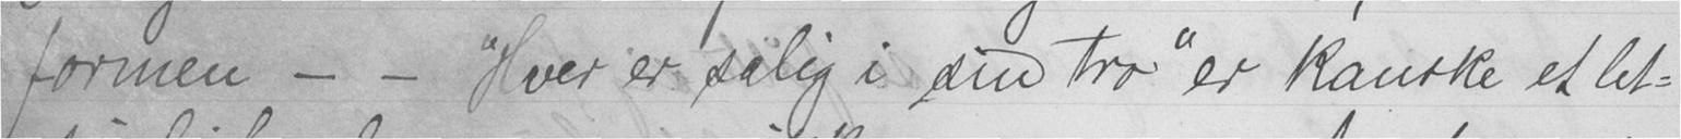formen - - "Hver er salig i sin tro" er kanske et let-
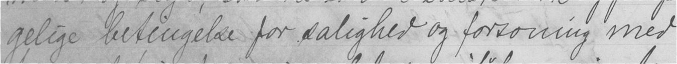gelige betingelse for salighed og forsoning med
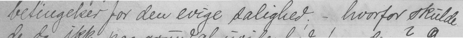betingelser for den evige salighed; - hvorfor skulde
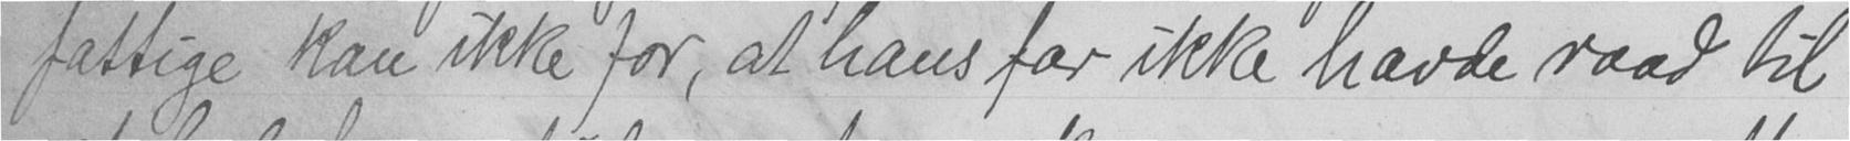fattige kan ikke for, at hans far ikke havde raad til
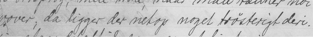over; da ligger der netop noget trøsterigt deri.
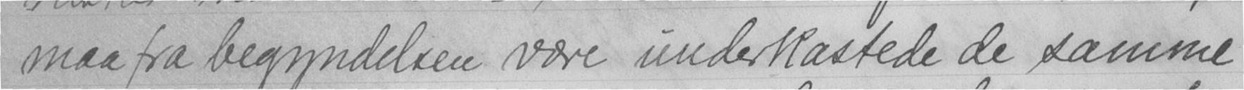maa fra begyndelsen være underkastede de samme
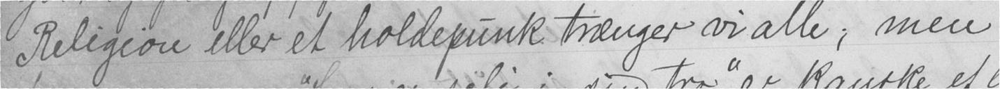Religion eller et holdepunk trænger vi alle; men
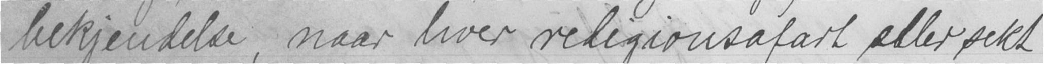bekjendelse, naar hver redigionsafart eller sekt
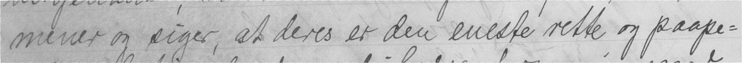mener og siger, at deres er den eneste rette og paape-
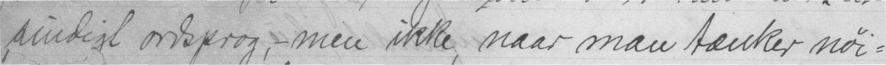sindigt ordsprog, - men ikke, naar man tænker nøi-
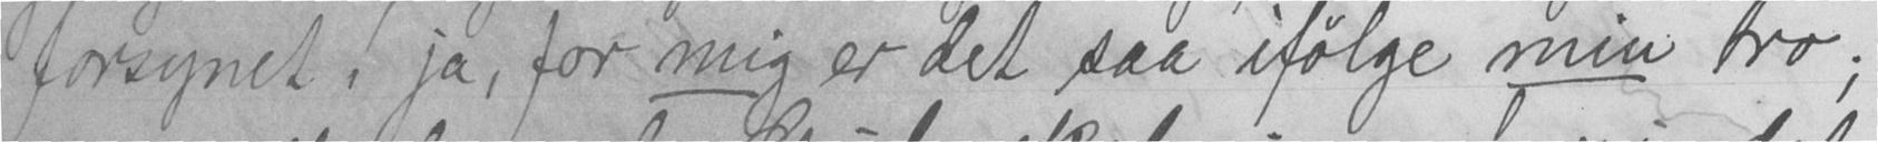forsynet! ja, for mig er det saa ifølge min tro;
